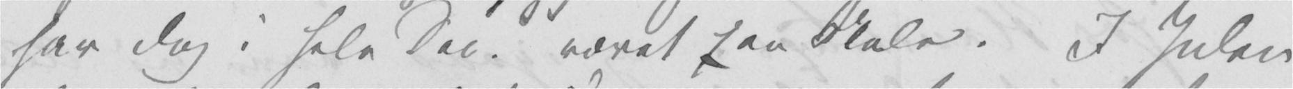har dog i hele Dec. været paa Skole. I Julen
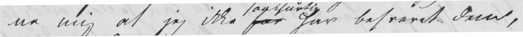ne mig at jeg ikke har har besvaret dem,
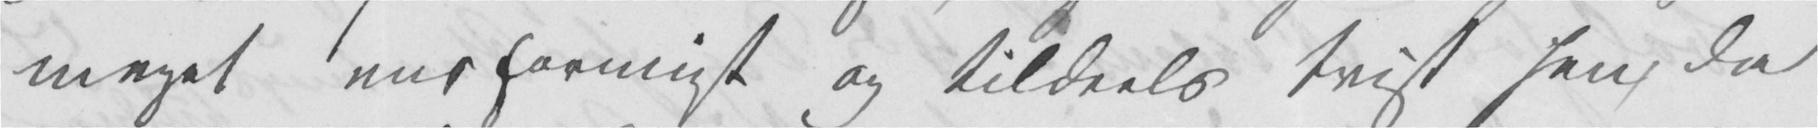meget ensformigt og tildeels trist hen, da
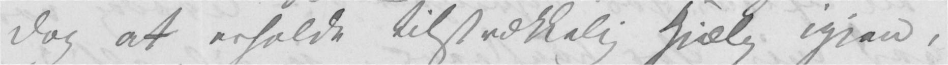dog at erholde tilstrækkelig Hjælp igjen,
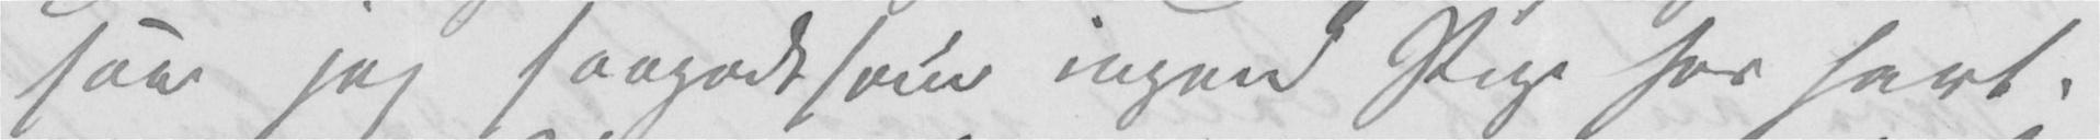saa jeg saagodtsom ingen Pige har havt.
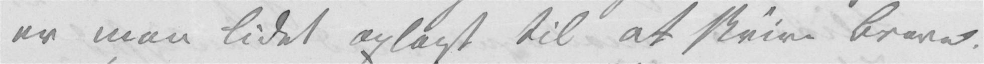er man lidet oplagt til at skrive Breve.
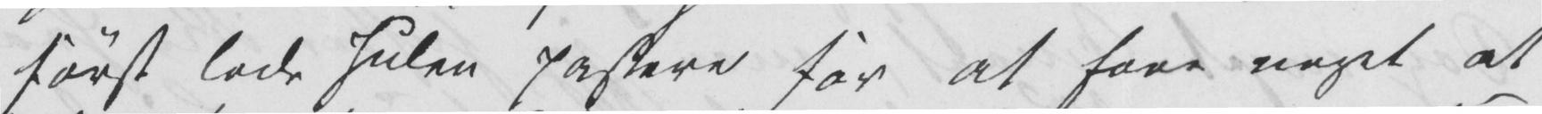først lade Julen passere for at faae noget at
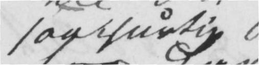saa hurtig
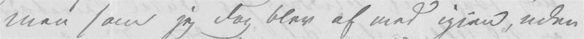men som jeg dog blev af med igjen, uden
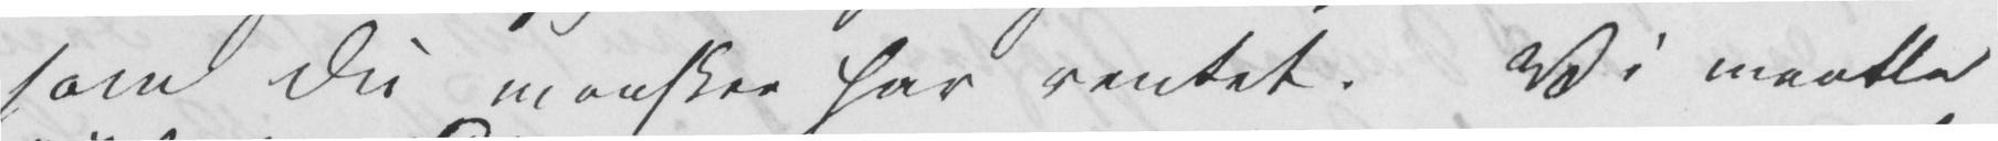som Du maaskee har ventet. Vi maatte
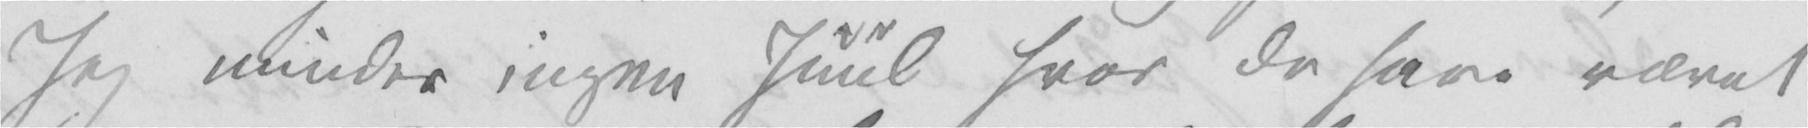Jeg mindes ingen Juul hvor de have været
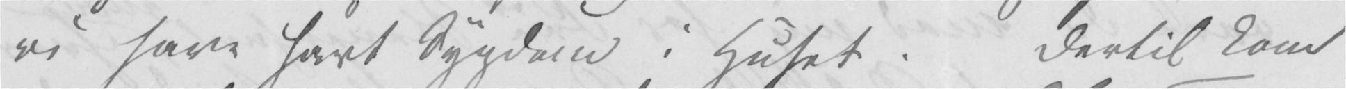vi have havt Sygdom i Huset. Dertil kom
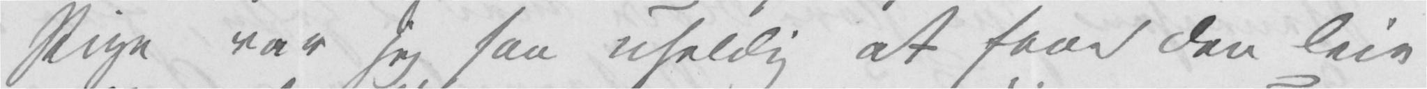Pige var jeg saa uheldig at faae den leie
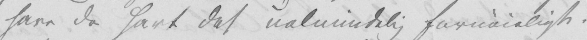have de havt det ualmindelig fornøieligt.
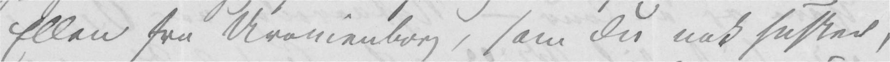Ellen fra Uranienborg, som Du nok husker,
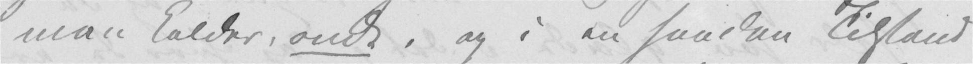man kalder, ondt, og i en saadan Tilstand
